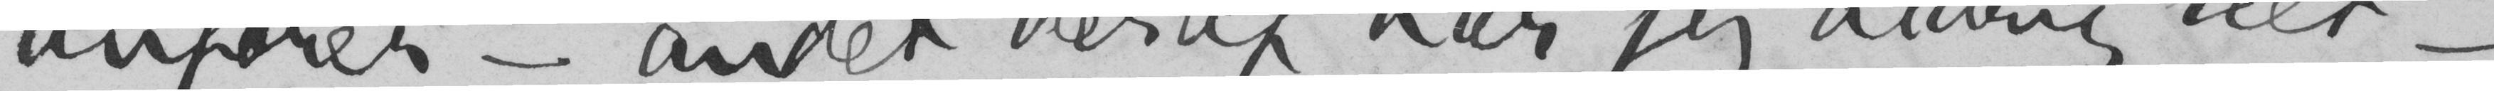anfører - andet deraf har jeg aldrig seet -
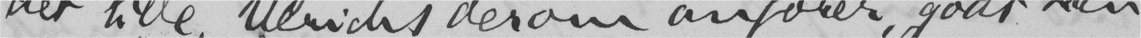det lille Ulrichs derom anfører, godt kan
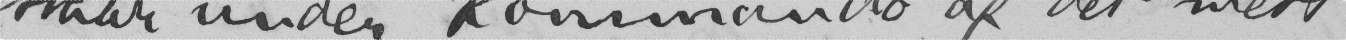staar under kommando af det mest
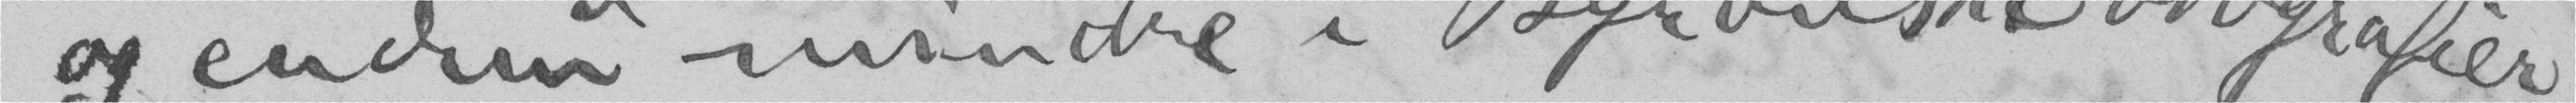og endnu mindre i Byronske biografier.
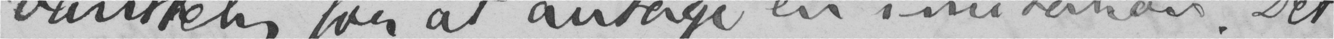vanskelig for at antage en imitiation. Det
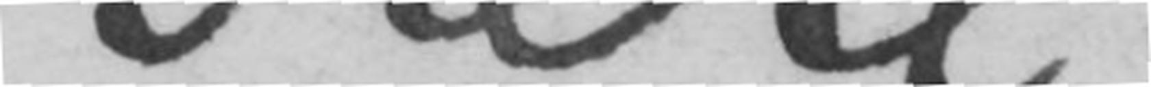være
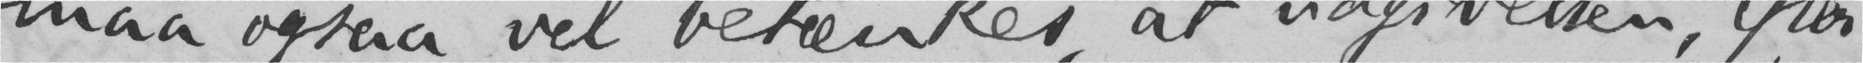maa ogsaa vel betænkes, at udgivelsen, efter
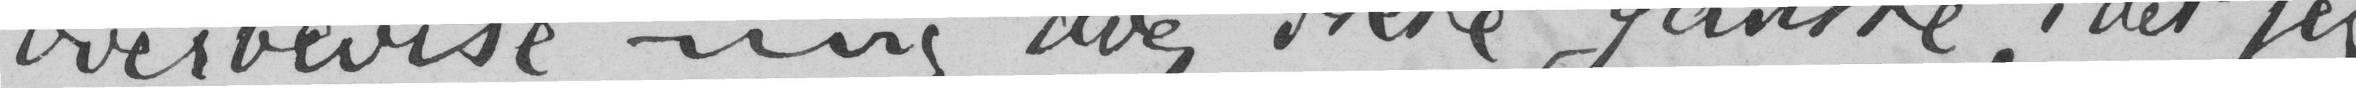overbevise mig dog ikke ganske, idet jeg
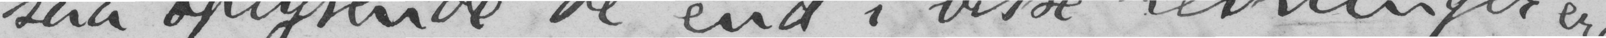saa oplysende de end i visse retninger ere,
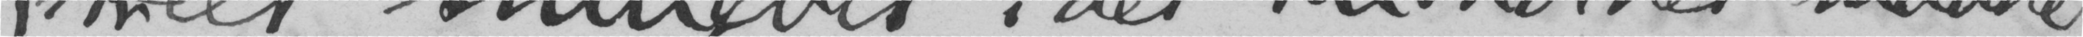skeet smugvis, idet indholdet maatte
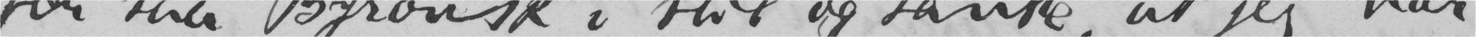for saa Byronsk i stil og tanke, at jeg har
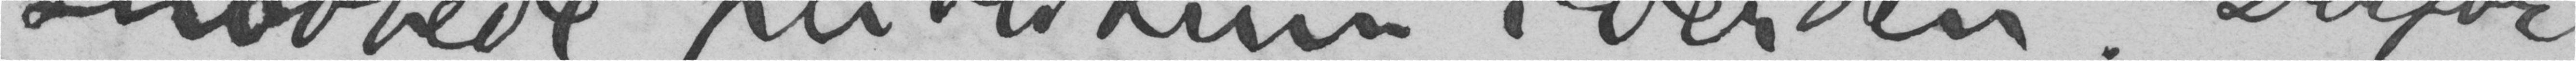snobbede publikum i verden. Derfor
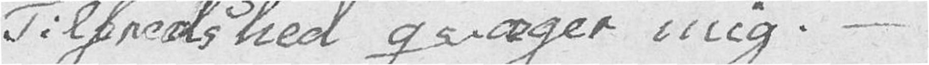Tilfredshed qvæger mig. –
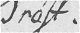Trøst.
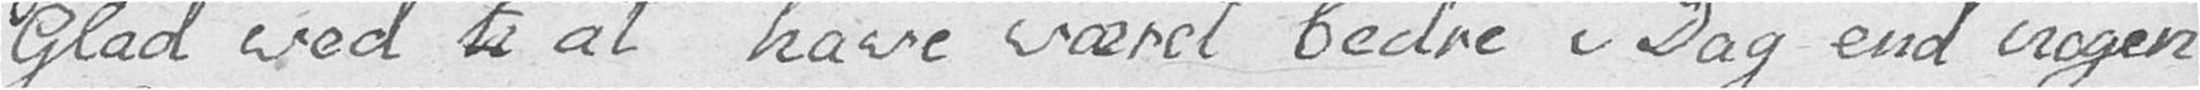Glad ved h at have været bedre i Dag end nogen
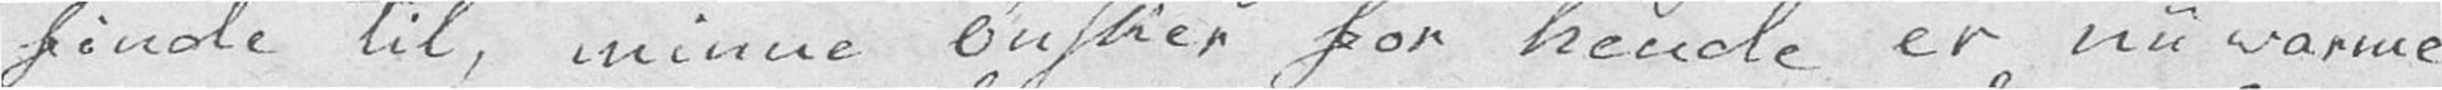finde til, minne ønsker for hende er nu varme
